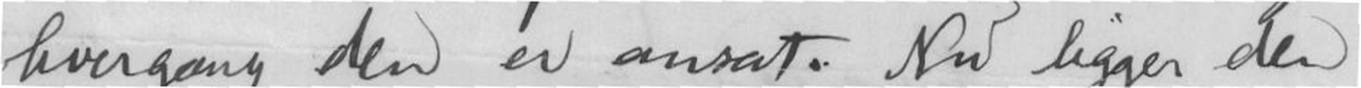hvergang den er ansat. Nu ligger der
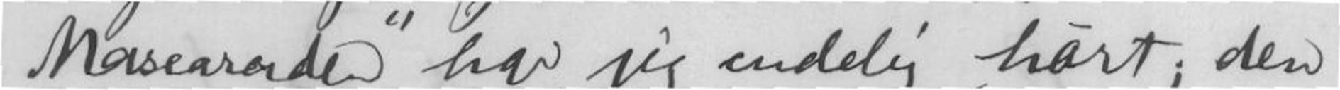Masceraden har jeg endelig hørt; den
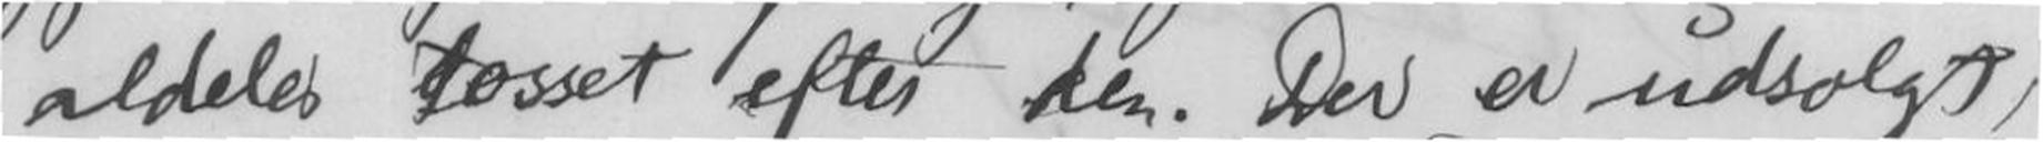aldeles tosset efter den. Der er udsolgt,
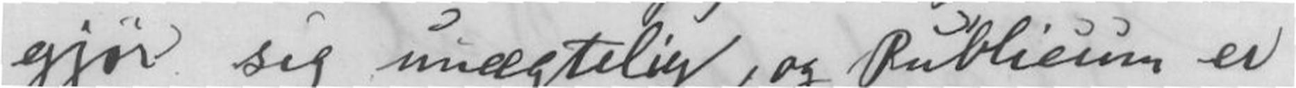gjør sig unægtelig, og Publicum er
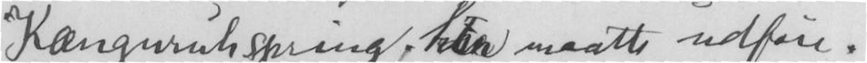Kænguruhspring, han maatte udføre.
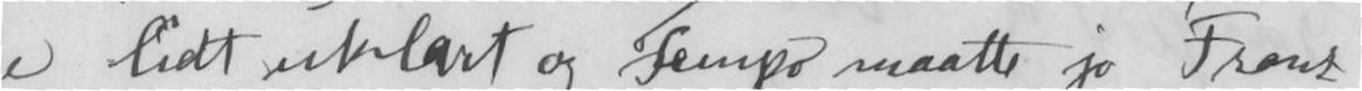lidt uklart og Tempo maatte jo Franz
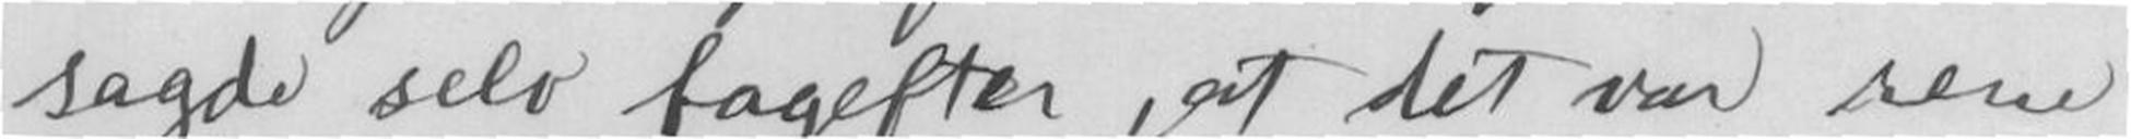sagde selv bagefter at det var rene
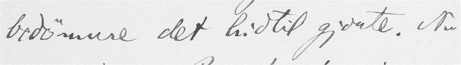bedømme det hidtil gjorte. Nu
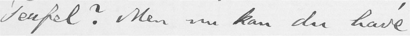Teufel? Men nu kan du have
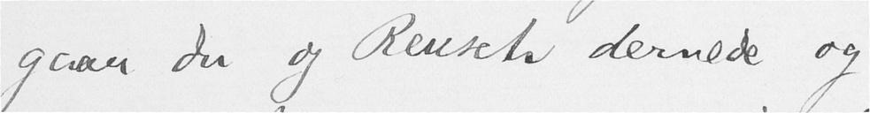gaar du og Reusch dernede og
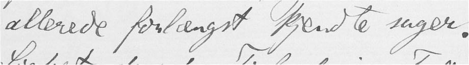allerede forlængst kjendte sager.
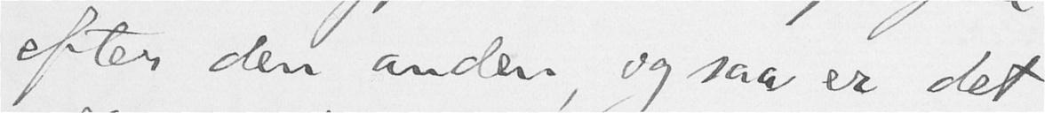efter den anden, og saa er det
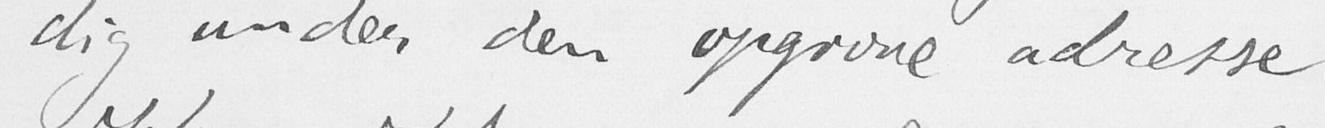dig under den opgivne adresse
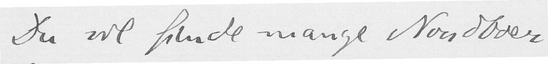Du vil finde mange Nordboer
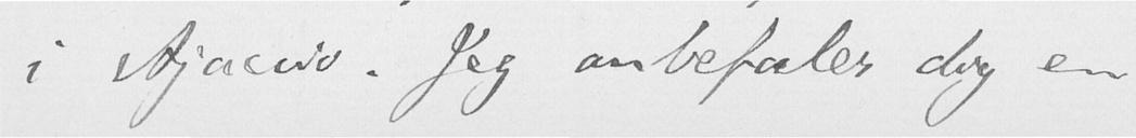i Ajaccio. Jeg anbefaler dog en
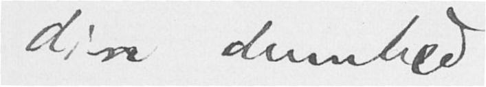din dumhed. -
